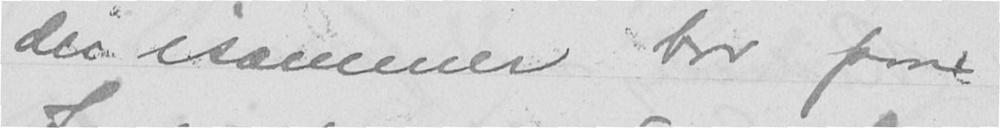der isommer bor paa
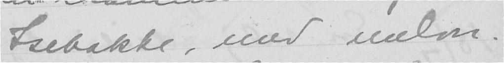Isebakke, med melon.
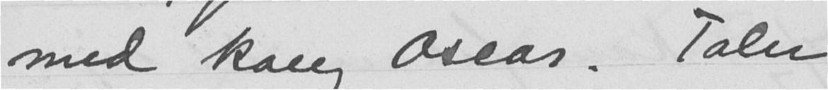med kong Oscar. Taler
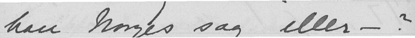han Norges sag, eller - ?
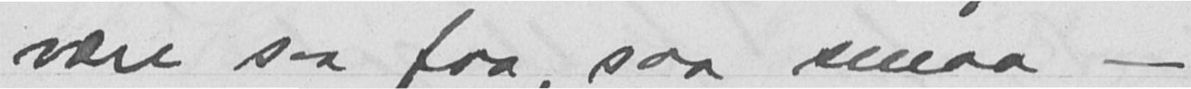være saa faa, saa smaa -
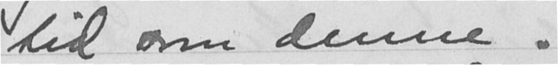tid som denne.
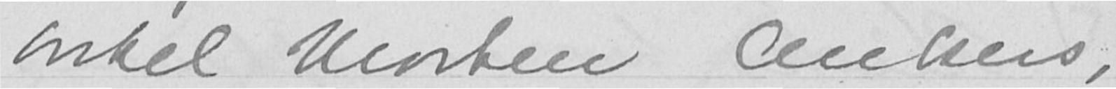onkel Morten Ankers,
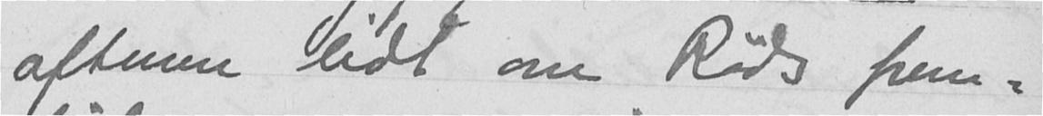aftenen lidt om Røds frem-
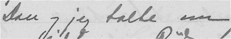Don og jeg talte om
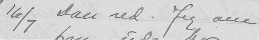16/7 Don red. Jeg om
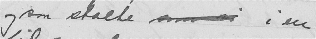og saa stolte som i i en
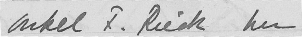Onkel F. Rieck her
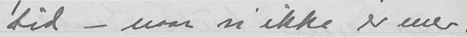tid - naar vi ikke er mer.
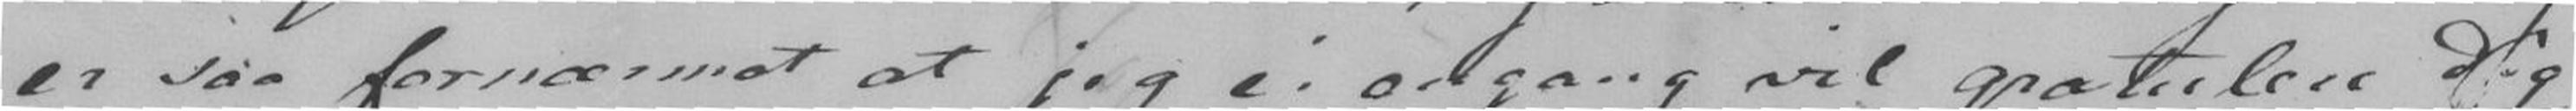er saa fornærmet at jeg ei engang vil gratulere Dig
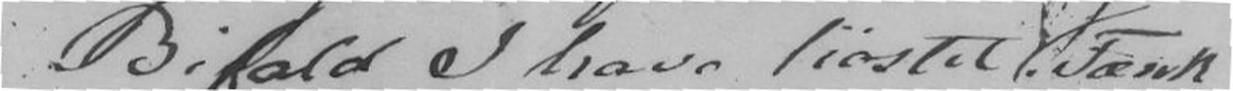Bifald I have høstet. Tænk
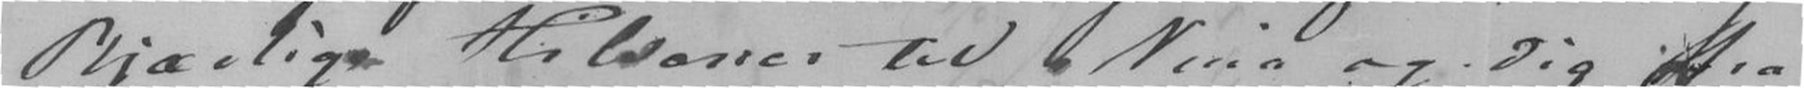kjærlige Hilsener til Nina og Dig fra
